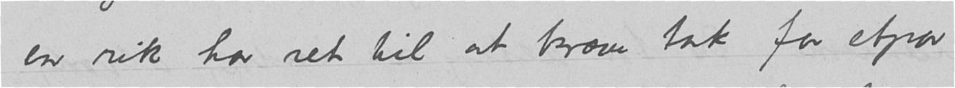en rik har ret til at kræve tak for etpar
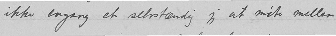ikke engang et selvstændig jeg at møte mellem
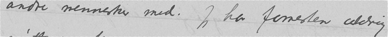andre mennesker med. Jeg har forresten aldrig
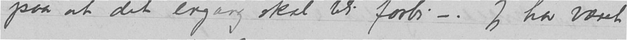paa at det engang skal bli forbi –. Jeg har været
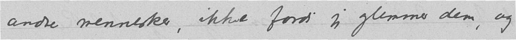andre mennesker, ikke fordi jeg glemmer dem, og
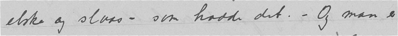elske og slaas – som hadde det. – Og man er
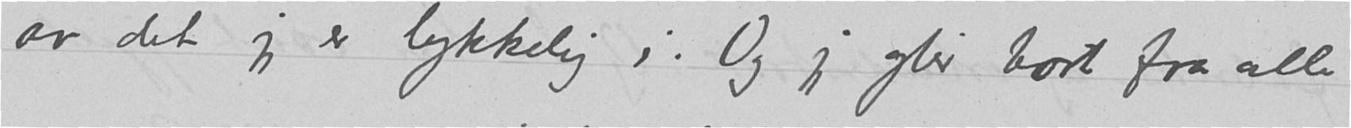av det jeg er lykkelig i. Og jeg glir bort fra alle
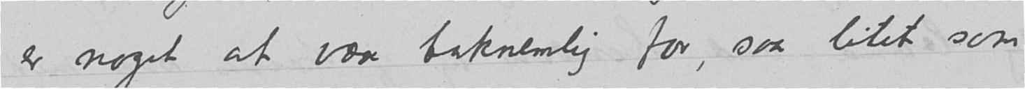er noget at være taknemlig for, saa litet som
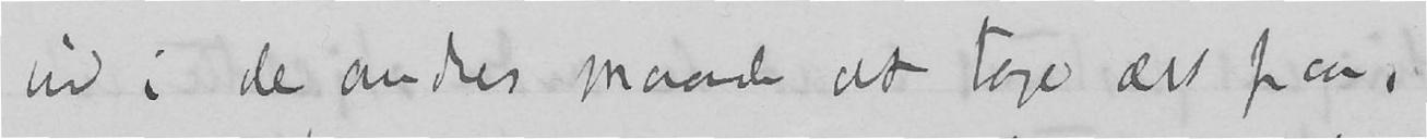ind i de andres maade at tage det paa,
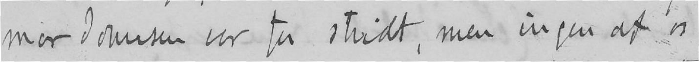mar Johnsen var for stridt, men ingen af os
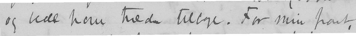og bedt ham træde tilbage. For min part,
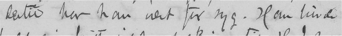dertil har han vært for syg. Han burde
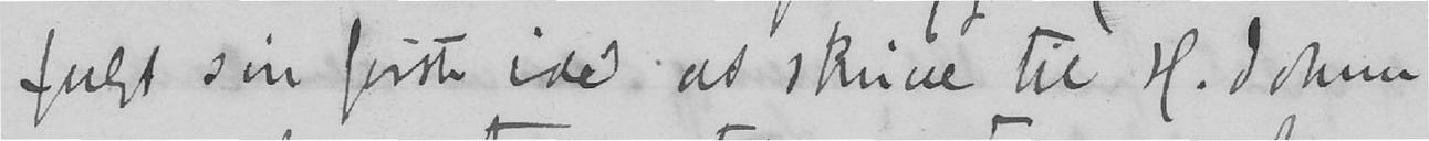fulgt sin første idé at skrive til H. Johnsen
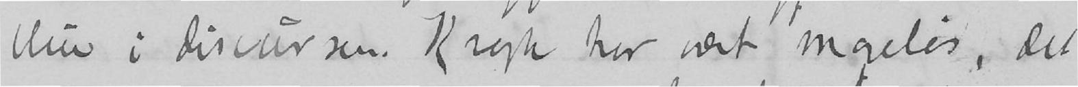blive i discursen. Krogh har vært mageløs, det
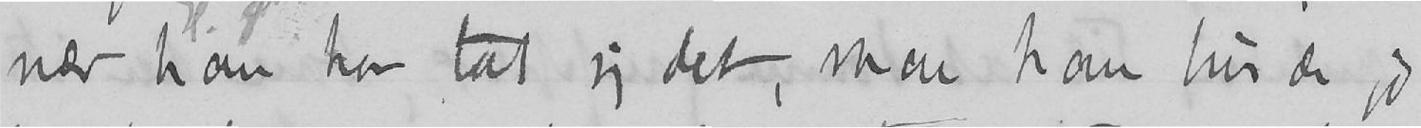nær han har tat sig det, men han kunde jo
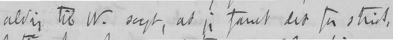aldrig til W. sagt, at jeg fandt det for stridt,
